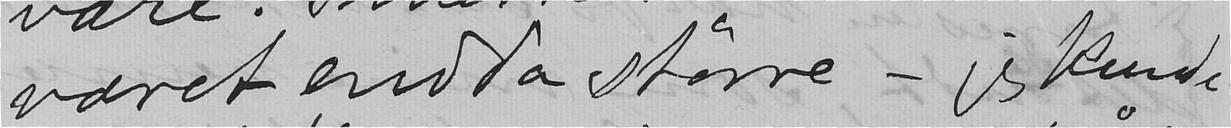været endda større - jeg kunde
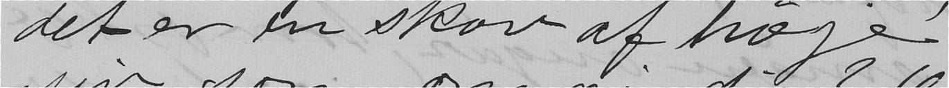det er en skov af høje
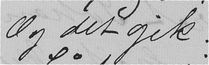Og det gik.
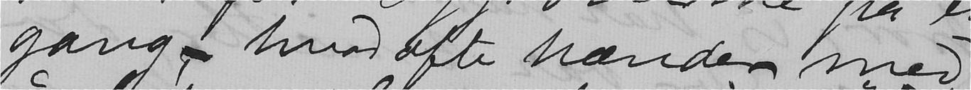gang, - hvad ofte hænder med
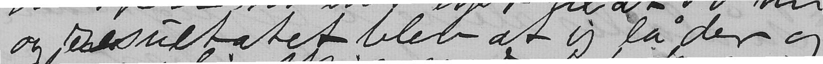og resultatet blev at jeg lå der og
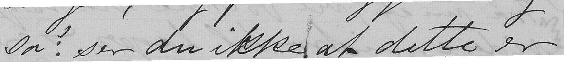sa: ser du ikke at dette er
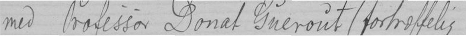med Professor Donat Guerout (fortræffelig
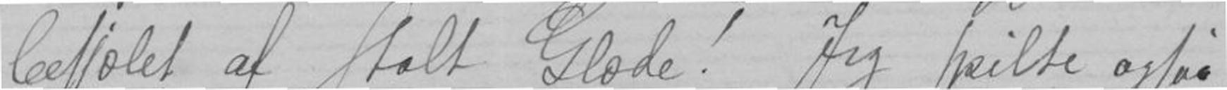besjælet af stolt Glæde! Jeg spilte ogsaa
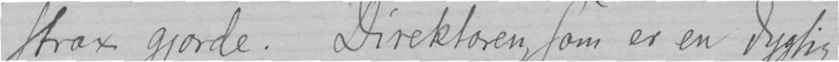strax gjorde. Direktoren som er en Dygtig
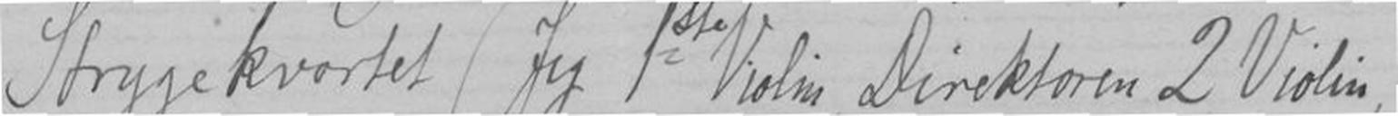Strygekvartet (Jeg 1ste Violin, Direktoren 2 Violin,
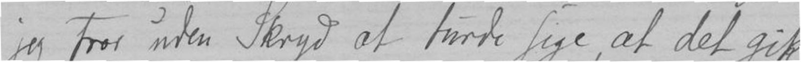jeg tror uden Skryd at turde sige, at det gik
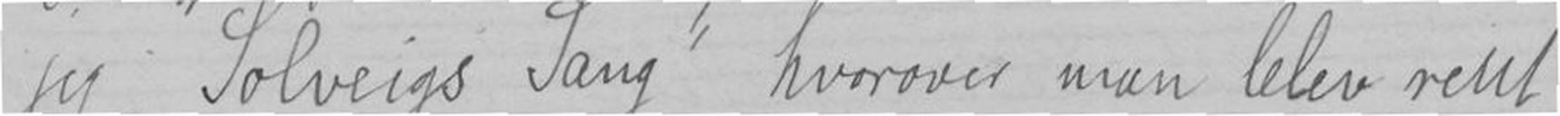jeg "Solveigs Sang" hvorover man blev rent
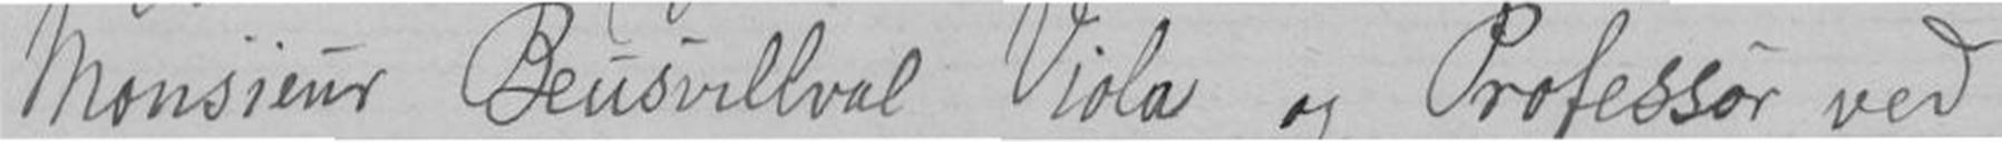Monsieur Beusvillval Viola og Professor ved
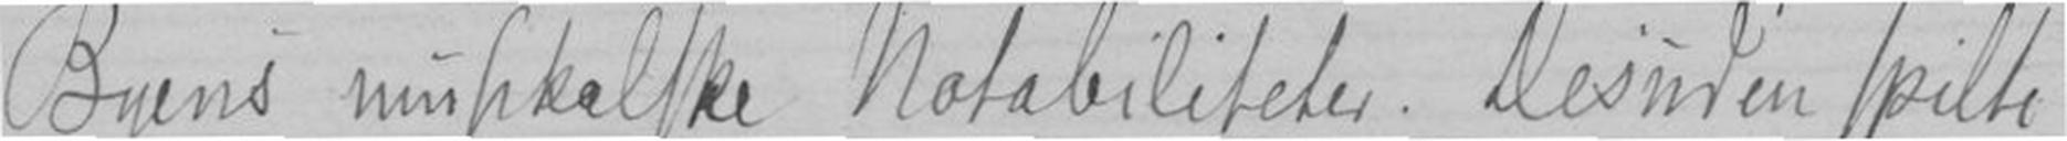Byens musikalske Notabiliteter. Desuden spilte
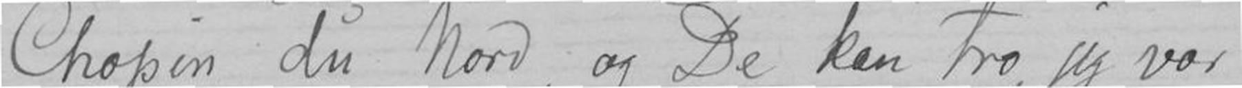Chopin du Nord, og De kan tro, jeg var
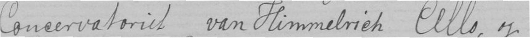Concervatoriet van Himmelrich Cello, og
